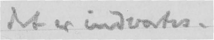det er indvortes.
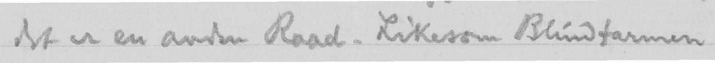det er en anden Raad. Likesom Blindtarmen
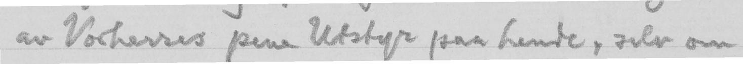av Vorherres pene Utstyr paa hende, selv om
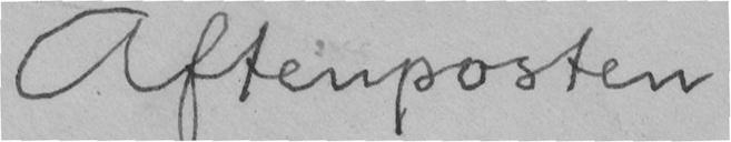Aftenposten
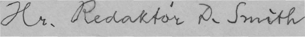Hr. Redaktør D. Smith
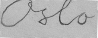Oslo
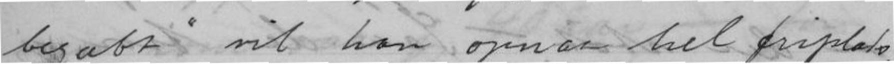begabt" vil han opnaa hel friplads,
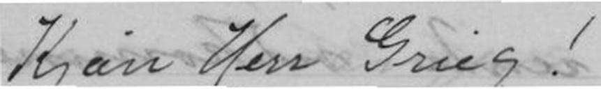Kjære Herr Grieg!
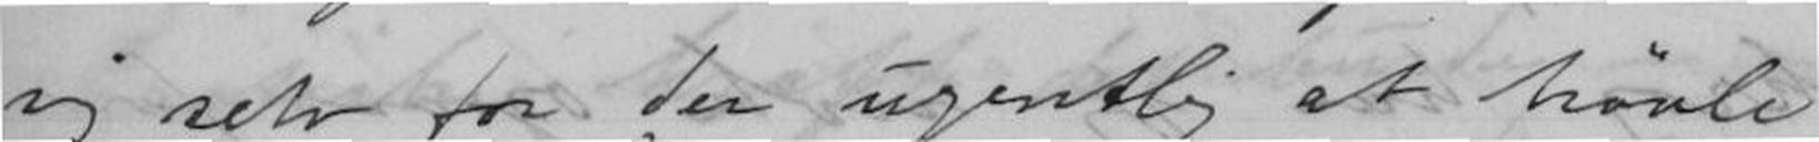sig selv for der ugentlig at høvle
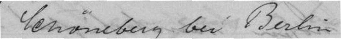Schøneberg bei Berlin
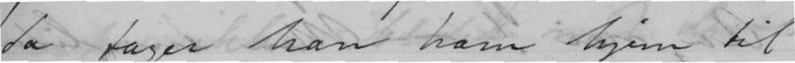da tager han ham hjem til
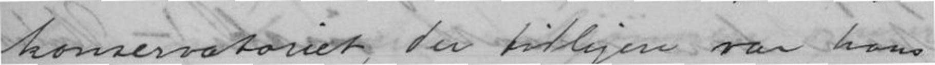konservatoriet, der tidligere var hans
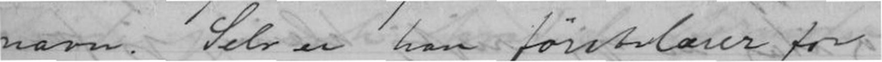navn. Selv er han førstelærer for
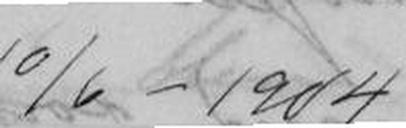10/6-1904
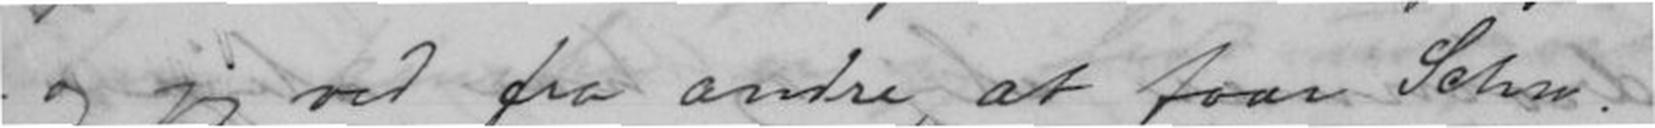- og jeg ved fra andre, at faar Schw.
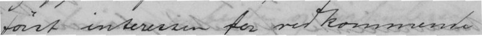først interessen for vedkommende
